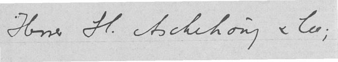Herrer H. Aschehoug & Co,
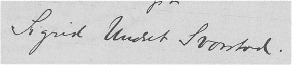Sigrid Undset Svarstad.
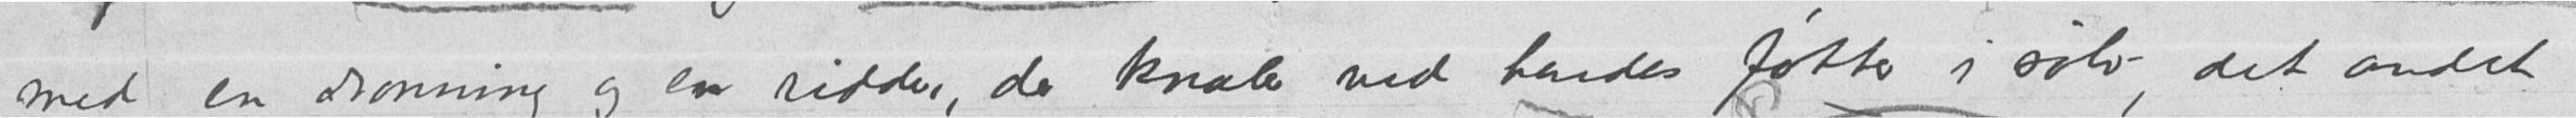med en dronning og en ridder, der knæler ved hendes føtter i sølv, det andet
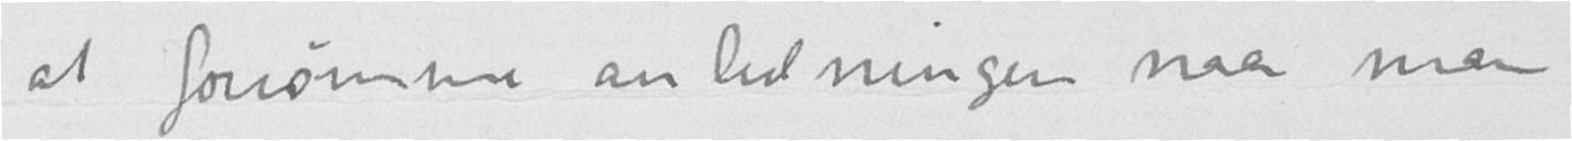at forsømme anledningen naar man
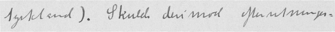Tyskland). Skulde derimod efterretninger-
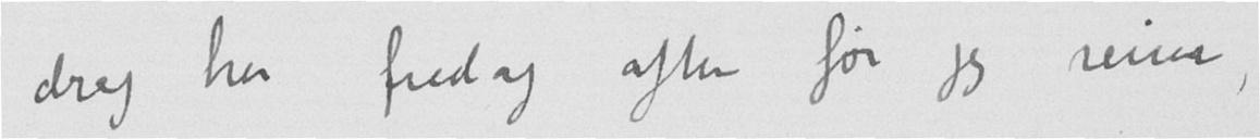drag her fredag aften før jeg reiser,
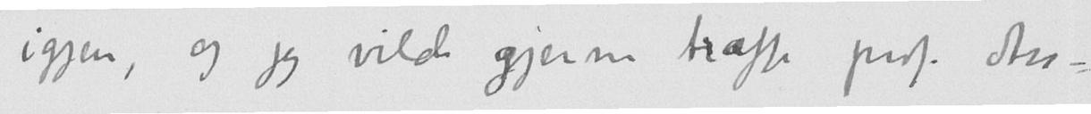igjen, og jeg vilde gjerne træffe prof. An-
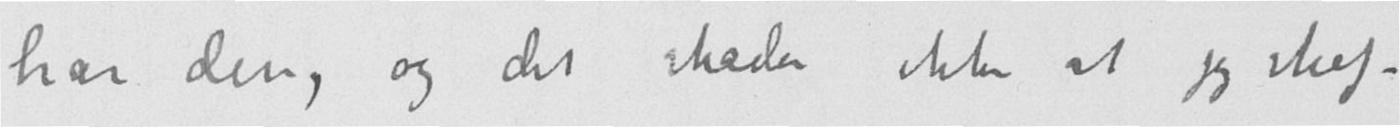har den, og det skader ikke at jeg skaf-
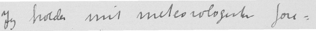Jeg holder mit meteorologiske fore-
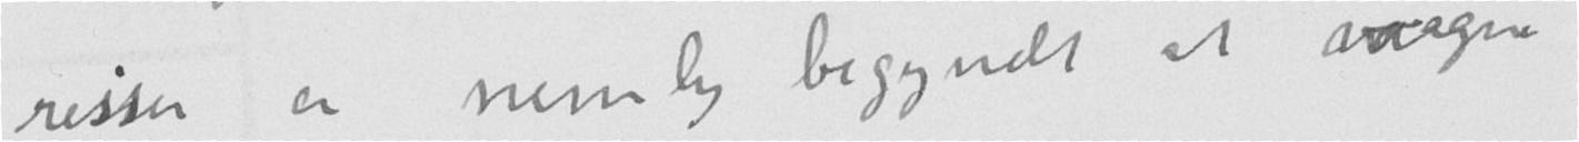resser er nemlig begyndt at vaagne
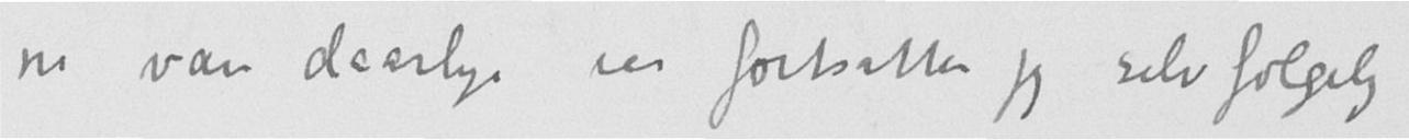ne være daarlige saa fortsætter jeg selvfølgelig
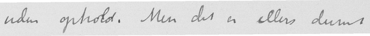uden ophold. Men det er ellers dumt
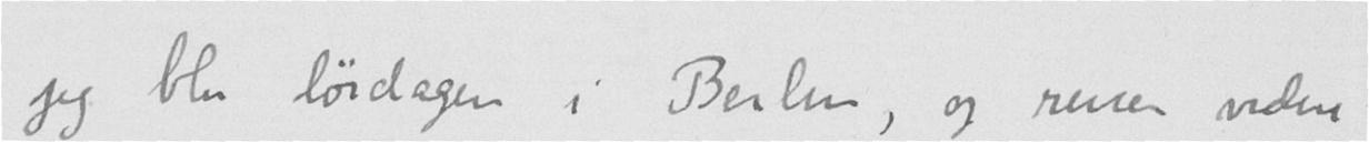jeg blir lørdagen i Berlin, og reiser videre
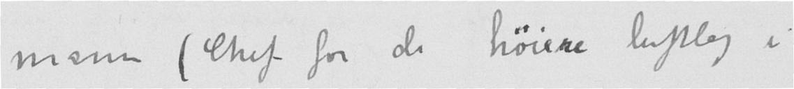mem (Chef for de høiere luftlag i
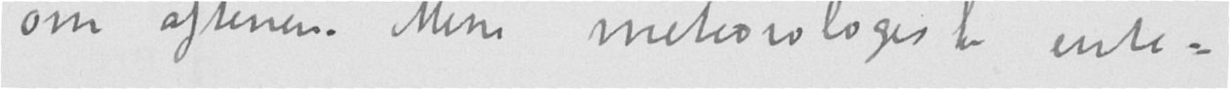om aftenen. Mine meteorologiske inte-
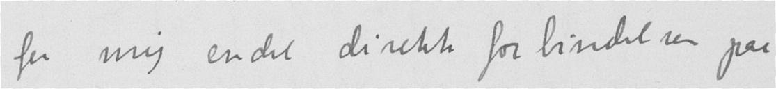fer mig endel direkte forbindelser paa
